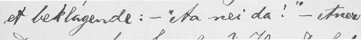et beklagende: – "Aa nei da!" – Aner
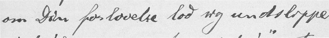om Din forlovelse lod sig undslippe
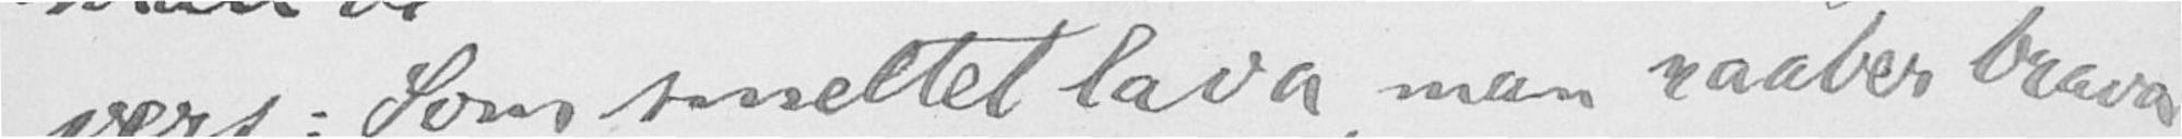vers: Som smeltet lava, man raaber bravo,
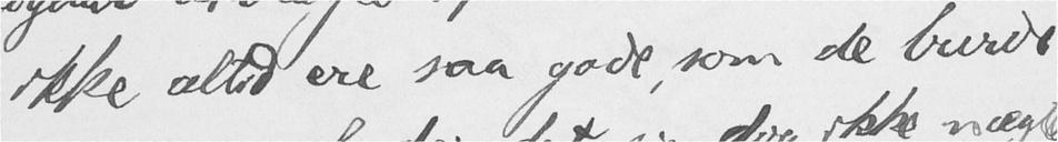ikke altid ere saa gode, som de burde
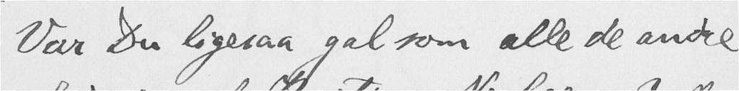Var Du ligesaa gal som alle de andre
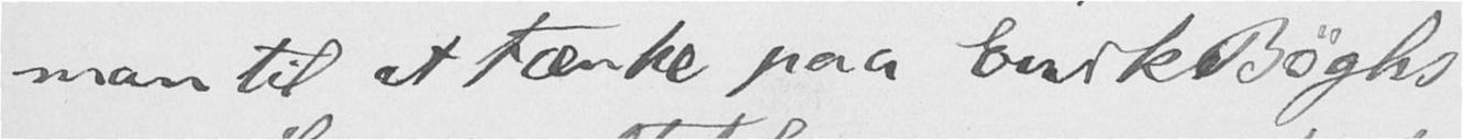man til at tænke paa Erik Bøghs
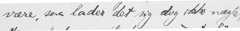være, saa lader det sig dog ikke nægte,
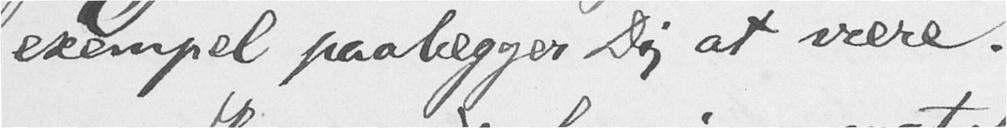exempel paalægger Dig at være.
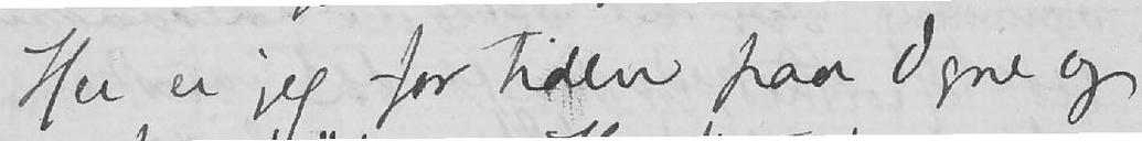Her er jeg for tiden paa Ogne og
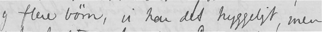og flere børn, vi har det hyggeligt, men
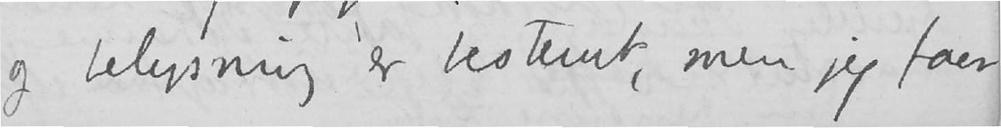og belysning er bestemt, men jeg faar
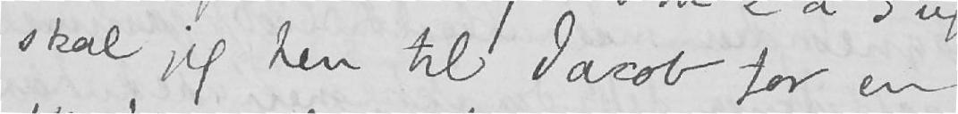skal jeg hen til Jacob for en
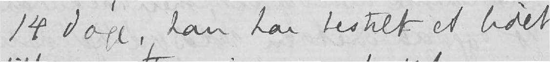14 dage, han har bestilt et lidet
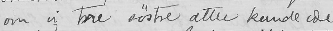om vi tre søstre atter kunde være
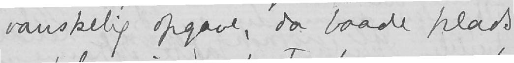vanskelig opgave, da baade plads
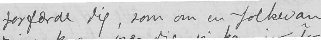forfærde dig, som om en folkevan-
